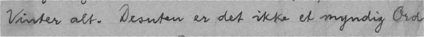Vinter alt. Desuten er det ikke et myndig Ord
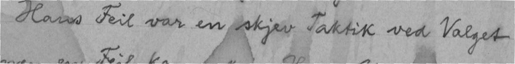Hans Feil var en skjev Taktik ved Valget
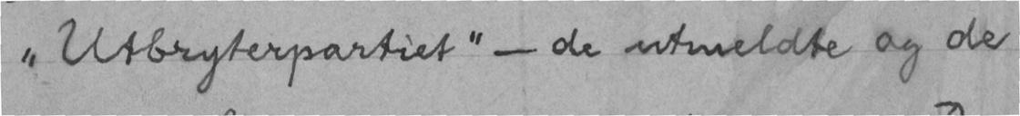"Utbryterpartiet" - de utmeldte og de
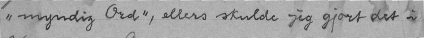"myndig Ord", ellers skulde jeg gjort det i
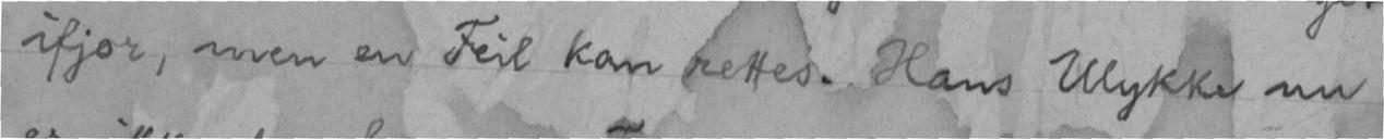ifjor, men en Feil kan rettes. Hans Ulykke nu
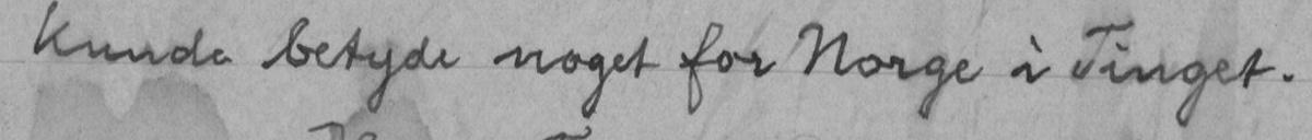kunde betyde noget for Norge i Tinget.
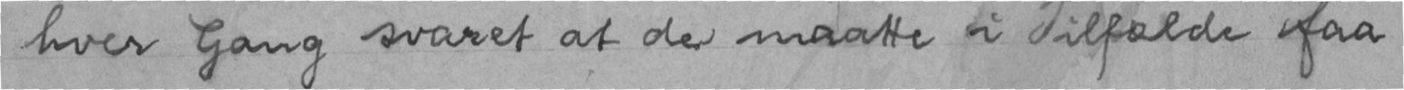hver Gang svaret at de maatte i Tilfælde faa
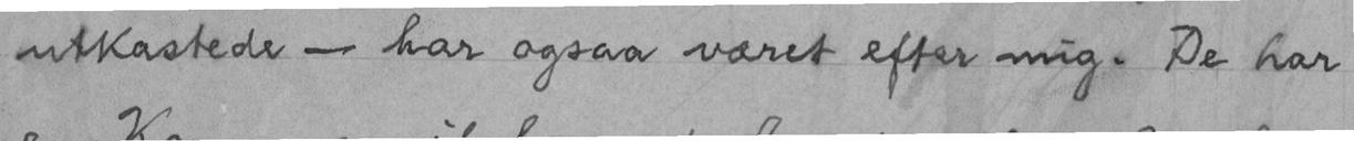utkastede - har ogsaa været efter mig. De har
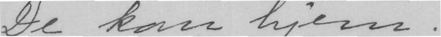De kom hjem.
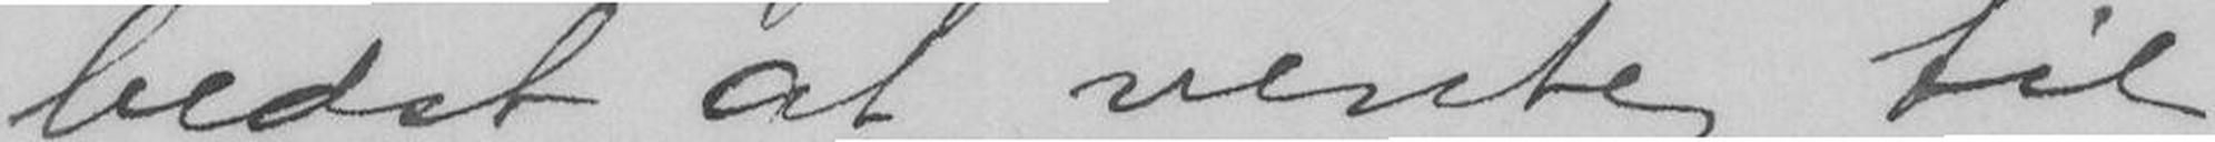bedst at vente til
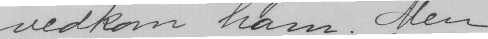vedkom ham. Men
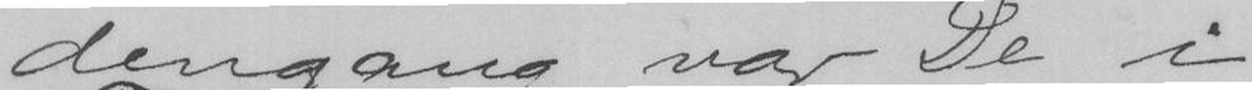dengang var De i
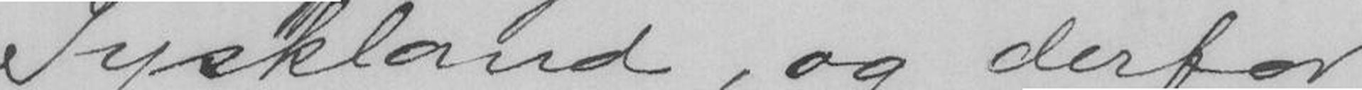Tyskland, og derfor
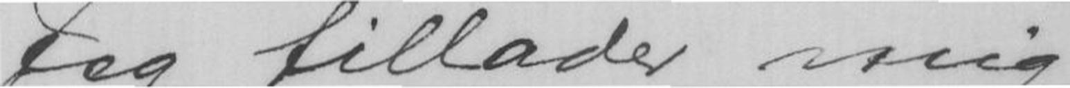Jeg tillader mig
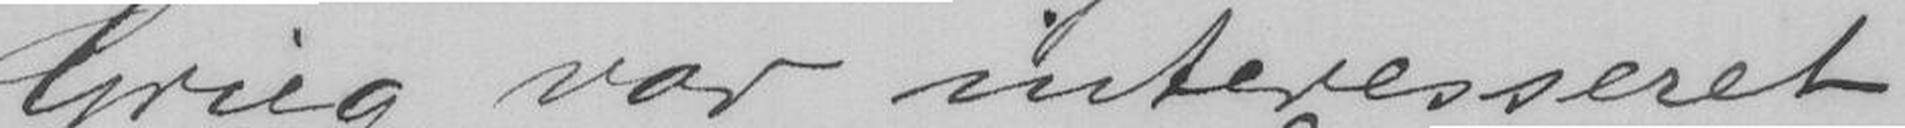Grieg var interesseret
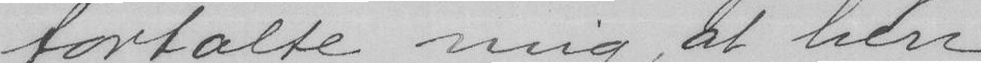fortalte mig, at herr
